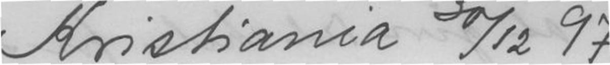Kristiania 30/12 97
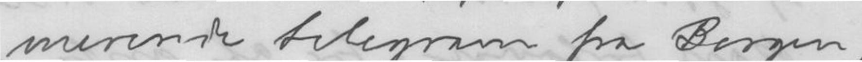merende telegram fra Bergen,
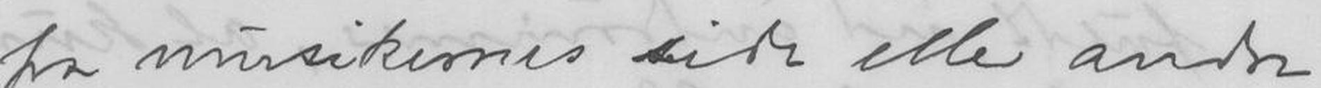fra musikernes side eller andre
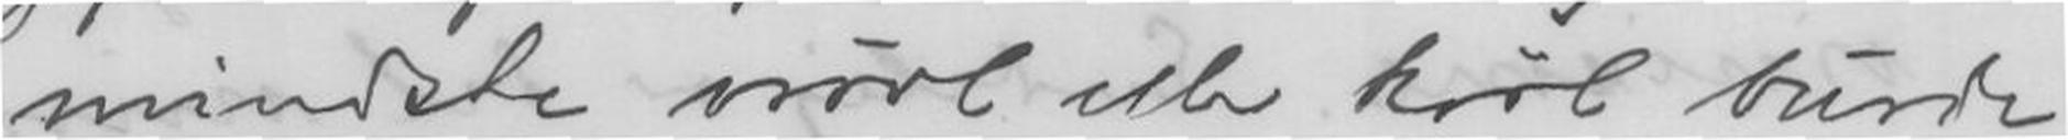mindste vrøvl eller krøl burde
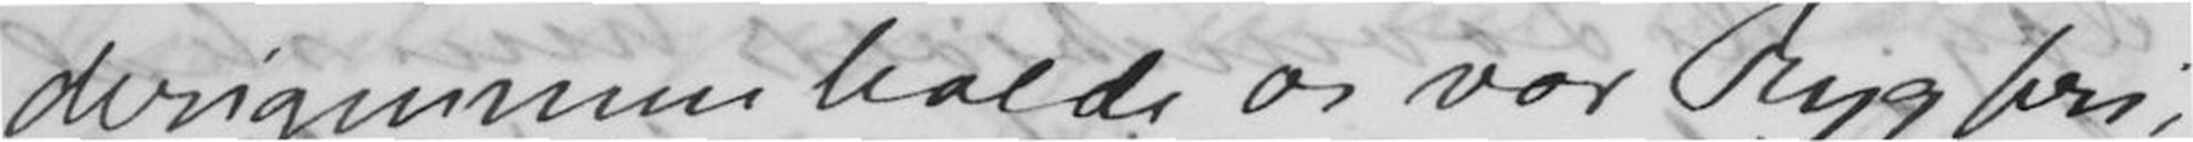derigennem holde os vor Ryg fri,
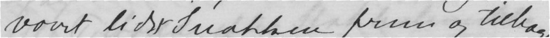vaeret lidt Snakken frem og tilbage
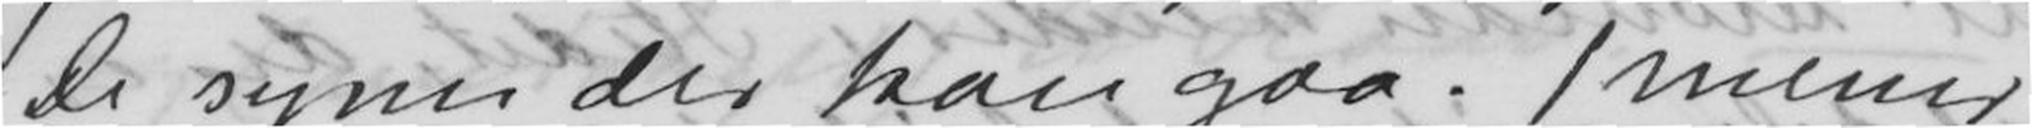De synes der kan gaa. J mener
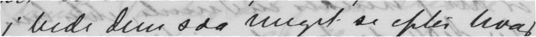j bede Dem saa meget se efter hvad
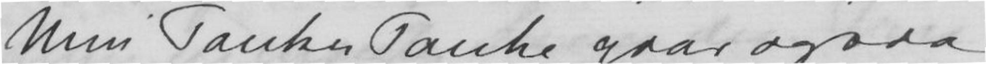Min Tankes Tanke gaar ogsaa
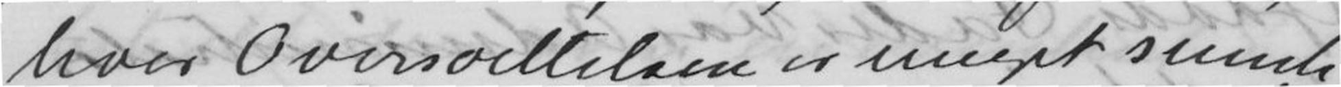hvor Oversaettelsen er meget smuk
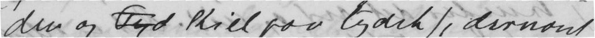den og Tyd Kiel paa Tydsk), dernaest
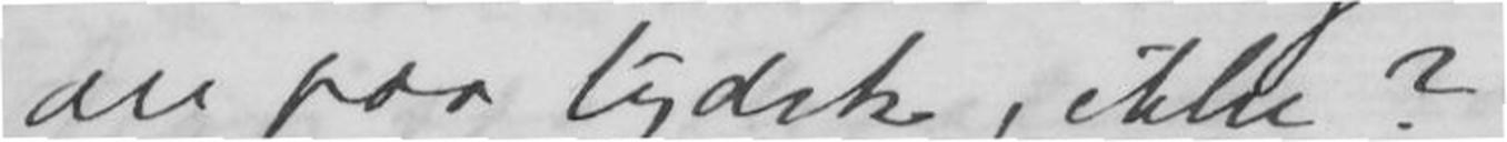an paa tydsk, ikke?
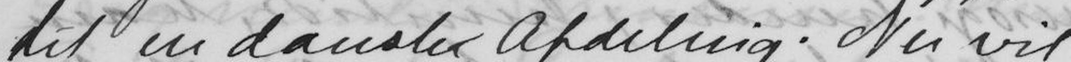til en dansk Afdeling. Nu vil
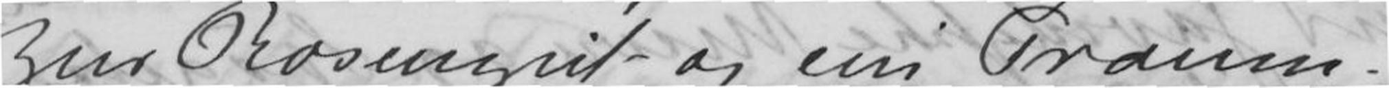Zur Rosenzeit og ein Traum.
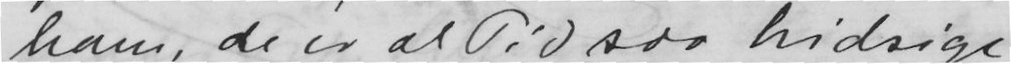ham, de er al Tid saa hidsige
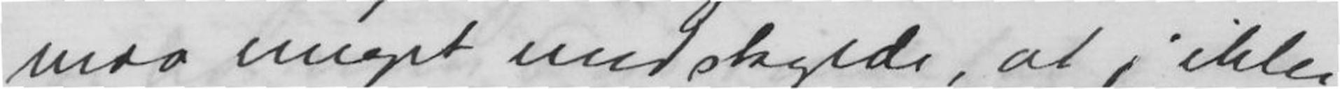maa meget undskylde, at j ikke
